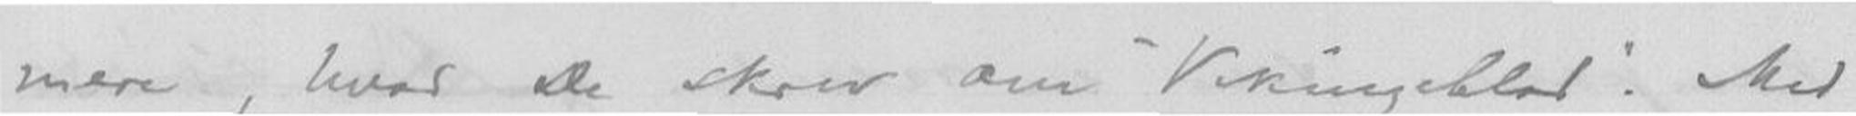mere, hvad De skrev om "Vikingblod". Med
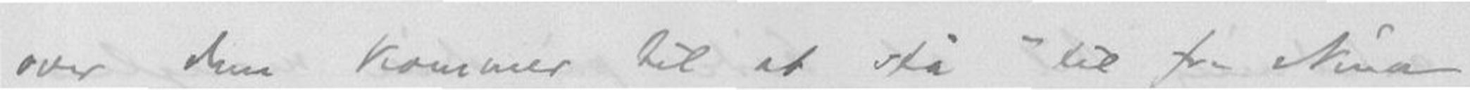over dem kommer til at stå "til fru Nina
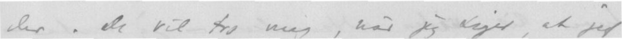der. De vil tro mig, når jeg siger, at jeg
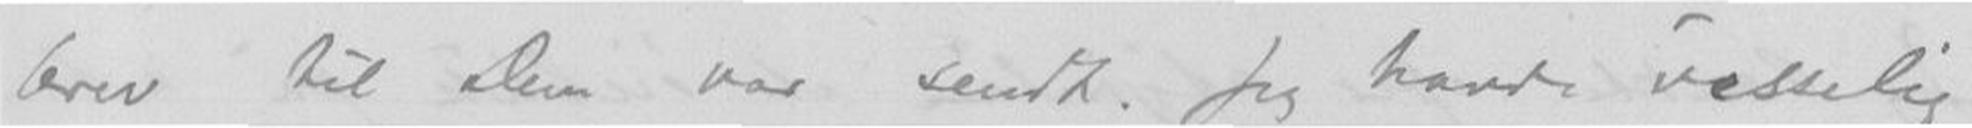brev til Dem var sendt. Jeg havde visselig
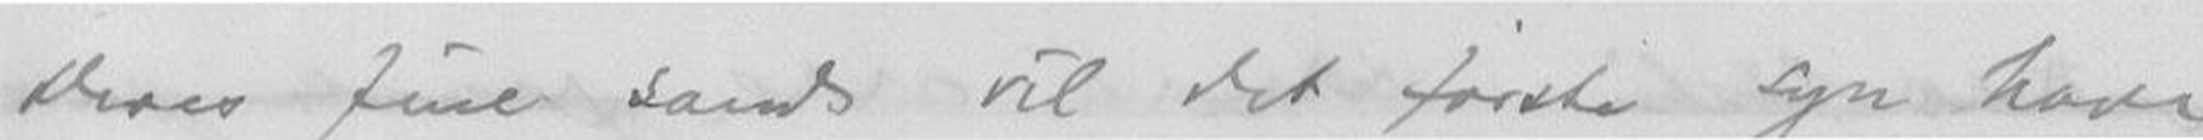Deres fine sands vil det første syn have
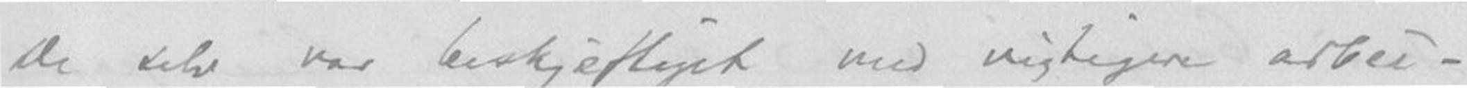De selv var beskjeftiget med vigtigere arbei-
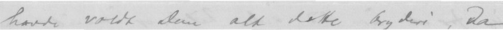havde voldt Dem alt dette bryderi, da
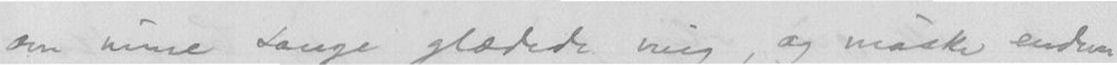om mine sange glædede mig, og måske endnu
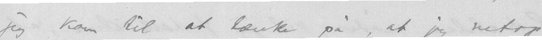jeg kom til at tænke på, at jeg netop
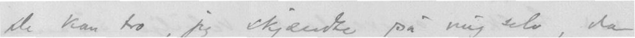De kan tro, jeg skjændte på mig selv, da
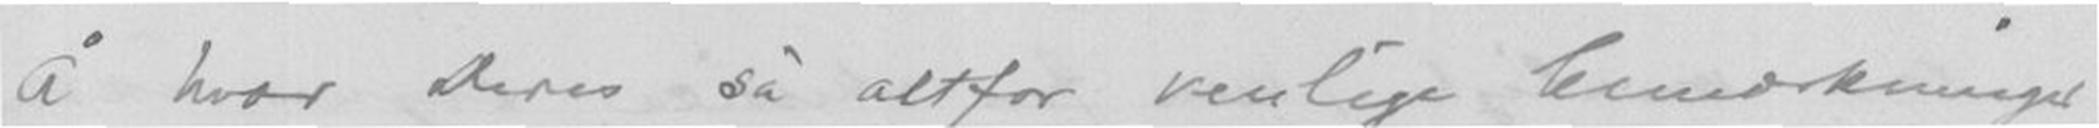Å hvor Deres så altfor venlige bemærkninger
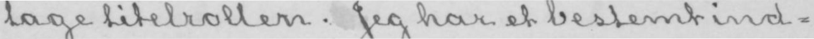tage titelrollen. Jeg har et bestemt ind-
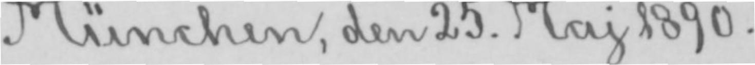München, den 25. Maj 1890.
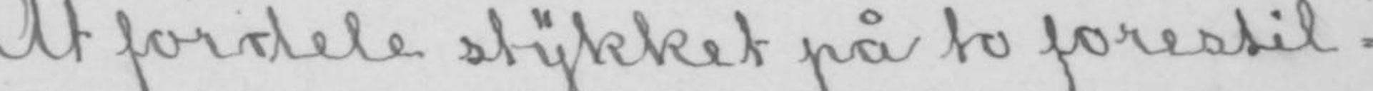At fordele stykket på to forestil-
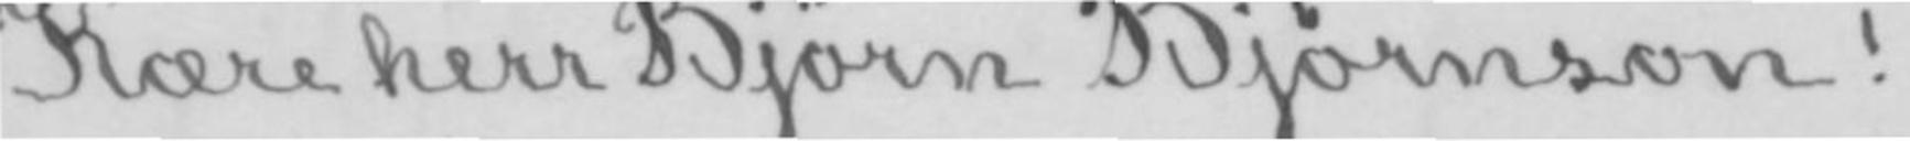Kære herr Bjørn Bjørnson!
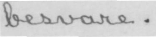besvare.
